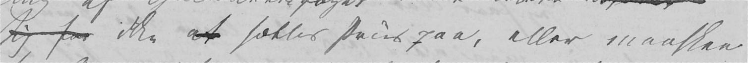sig for ikke at sættes Priis paa, eller maaske
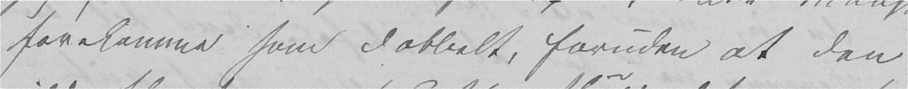forekomme som dobbelt, foruden at den
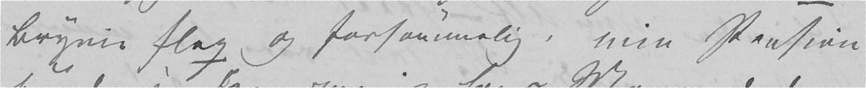Brynie slap og forsømmelig, min Pension
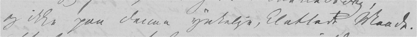og ikke paa denne ynkelige, klattede Maade.
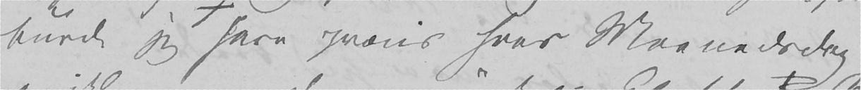burde jeg have præcis hver Maanedsdag,
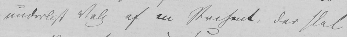underligt Valg af en Present, der skal
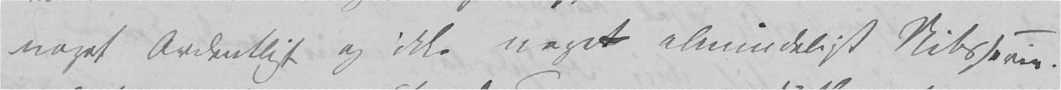noget Ordentligt og ikke noget almindeligt Nibsserie.
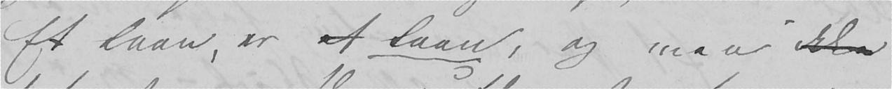Et Laan, er et Laan, og maa ikke
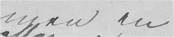maaskee
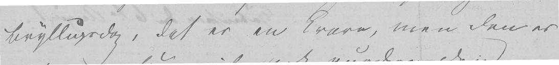Bryllupsdag, det er en Krave, men den er
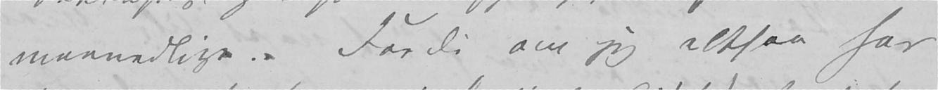maanedlige. Fordi om jeg altsaa har
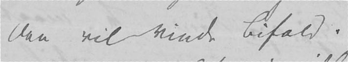den vil vinde Bifald.
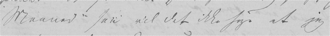Maaned saa vil det ikke sige at jeg
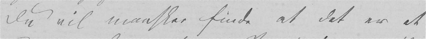Du vil maaskee finde at det er et
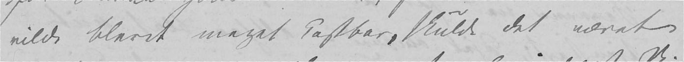vilde blevet meget kostbar, skulde det været
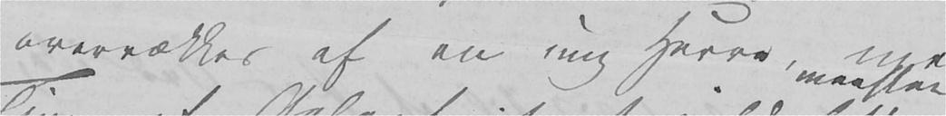overrækkes af en ung Herre, men en
